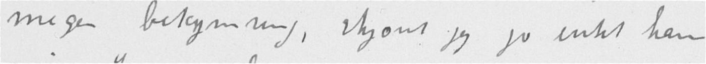megen bekymring, skjønt jeg jo intet kan
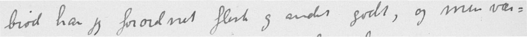brød har jeg forordnet flesk og andet godt, og min vær-
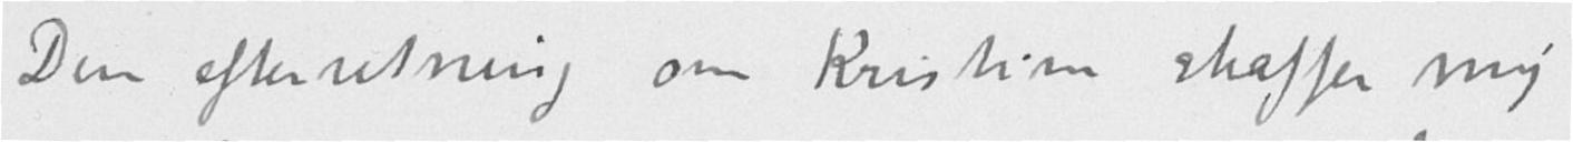Din efterretning om Kristine skaffer mig
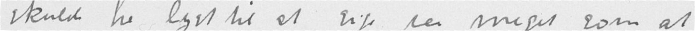skulde ha lyst til at sige saa meget som at
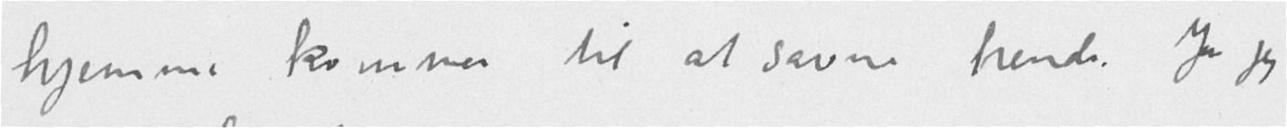hjemme kommer til at savne hende. Ja jeg
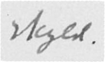skyld.
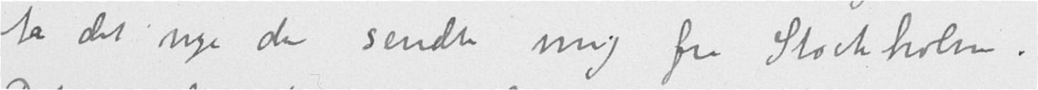ta det nye du sendte mig fra Stockholm.
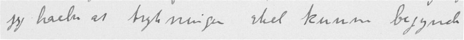jeg haaber at trykningen skal kunne begynde
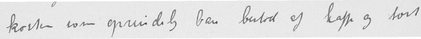kosten som oprindelig bare bestod af kaffe og tørt
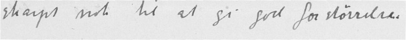skarpt nok til at gi god forstørrelse.
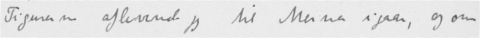Figurerne afleverede jeg til Meiner i gaar, og om
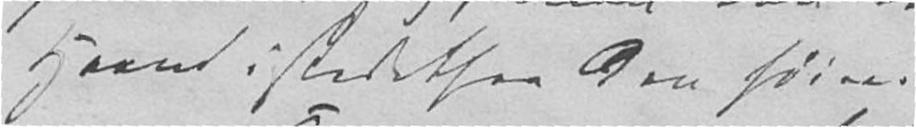Haand istedetfor den høire.
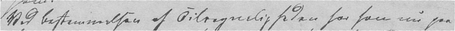Ved Bestemmelsen af Tilregneligheden har han nu paa-
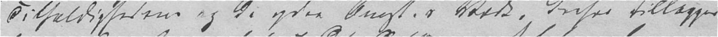Tilfældighedens og de ydre Omst.s Værk, derfor tillægger
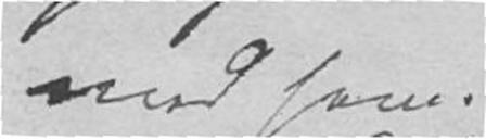ved ham.
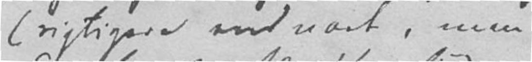(rigtigere end X, men
